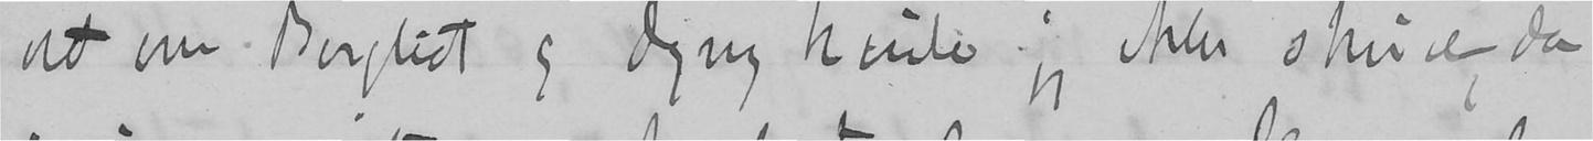at om Bergliot og Dagny kunde jeg ikke skrive, da
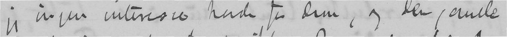jeg ingen interesse havde for dem, og den gamle
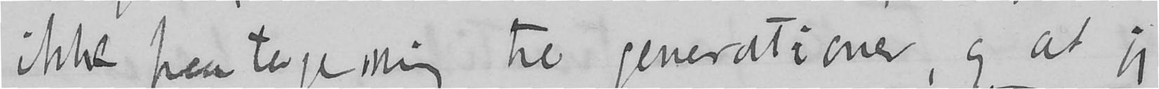ikke paatage mig tre generationer, og at jeg
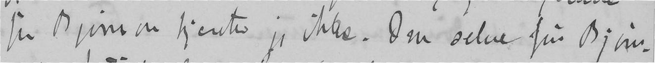fru Bjørnson kjendte jeg ikke. Om selve fru Bjørn-
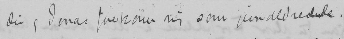du og Jonas forekom mig som jevnaldrende,
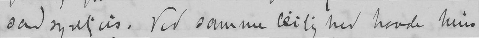sandsynligvis. Ved samme leilighed havde hun
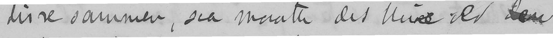disse sammen, saa maatte det blive ved den
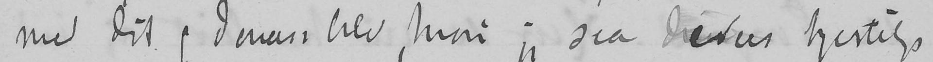med dit og Jonass brev, hvori jeg saa eders hjertelige
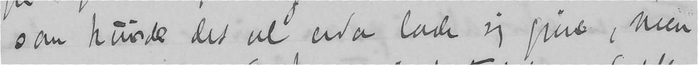son kunde det vel enda lade sig gjøre, men
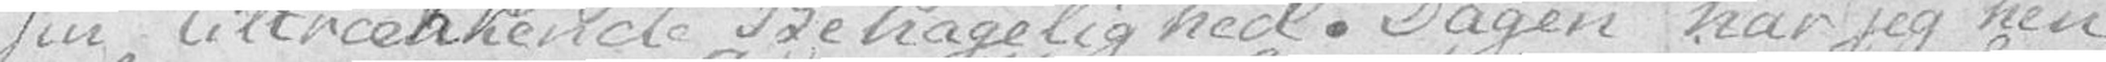sin tiltrækkende Behagelighed. Dagen har jeg hen
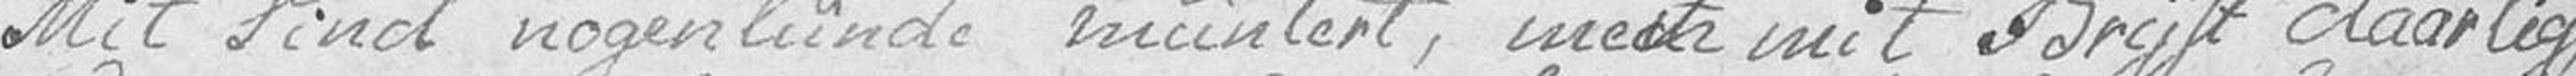Mit Sind nogenlunde muntert, men mit Bryst daarlig,
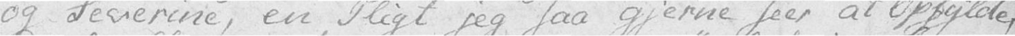og Severine, en Pligt jeg saa gjerne seer at Opfylde,
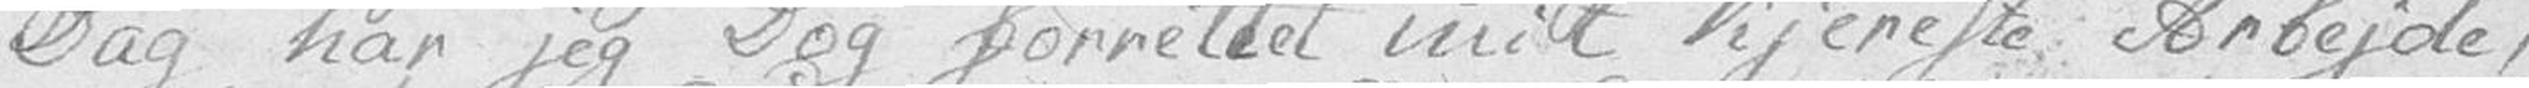Dag har jeg Dog forrettet mit kjereste Arbejde,
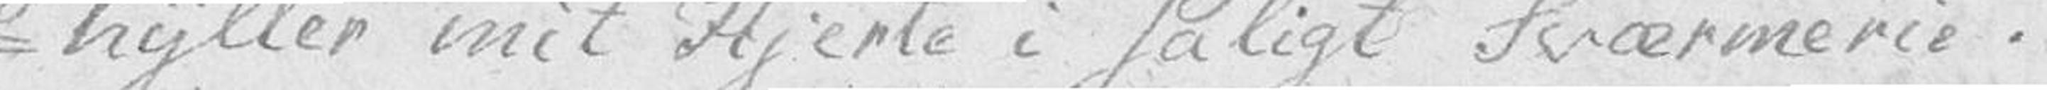-hyller mit Hjerte i saligt Sværmerie. –
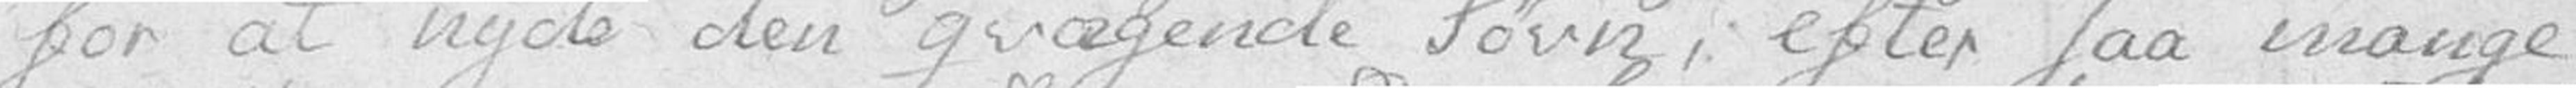for at nyde den qvægende Søvn, efter saa mange
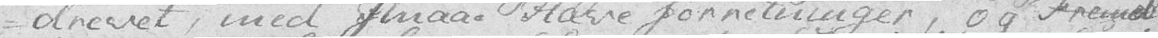-drevet, med smaa Have forretninger, og Fremd
